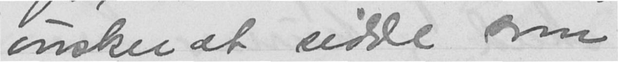ønsker at sidde som
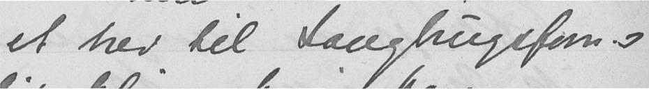et brev til Saugbrugsforn.s
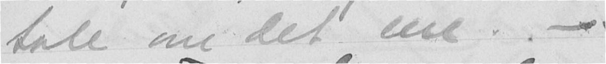tale om det ene. -
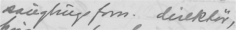saugbrugsforn. direktør,
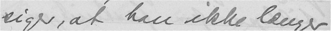siger, at han ikke længer
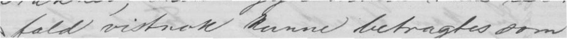fald vistnok kunne betragtes som
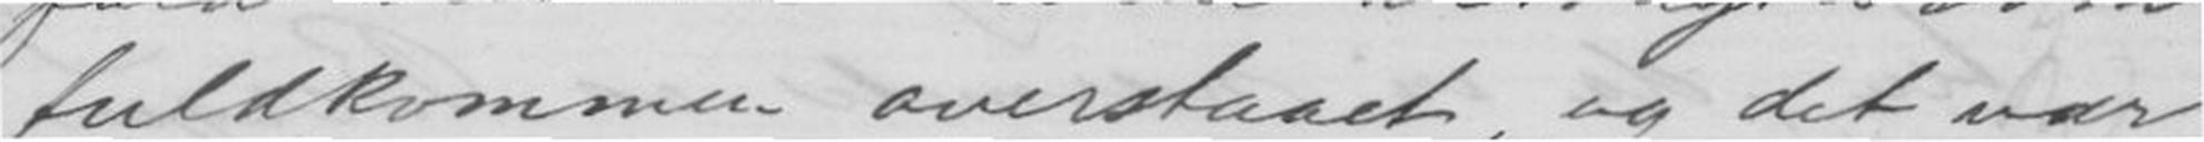fuldkommen overstaaet, og det var
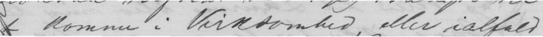at komme i Virksomhed, eller ialfald
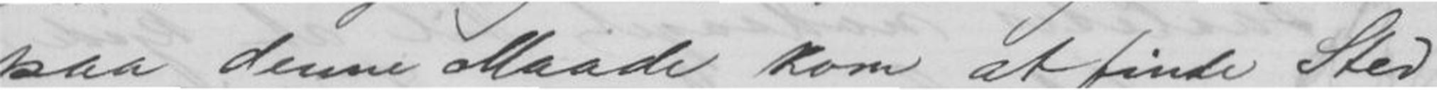paa denne Maade kom at finde Sted
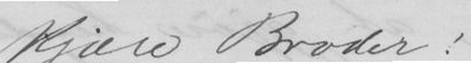Kjære Broder!
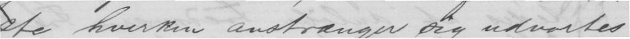ste hverken anstrænger sig udvortes
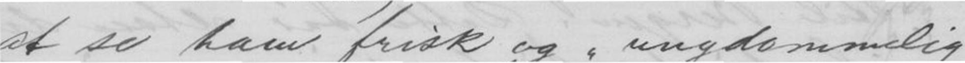at se ham frisk og ungdommelig
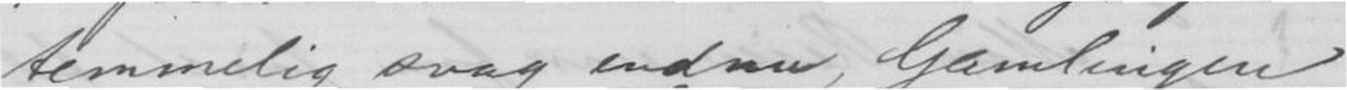temmelig svag endnu, Gamlingen
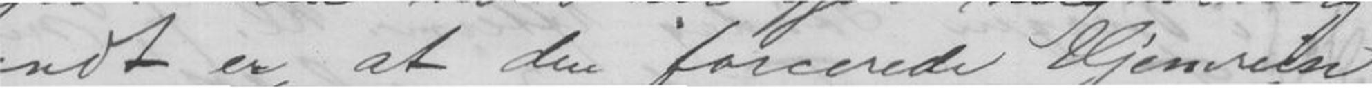ondt er at den forcerede Hjemreise
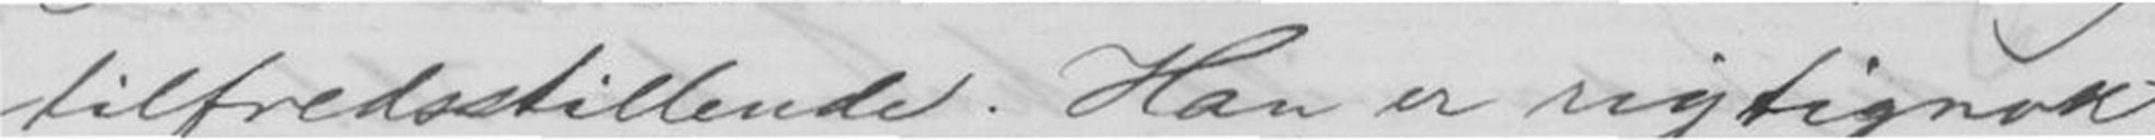tilfredsstillende. Han er rigtignok
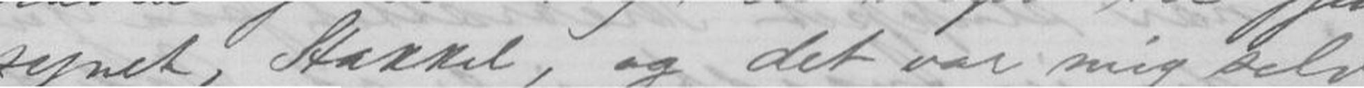synet, Stakkel, og det var mig selv
